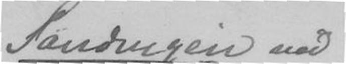Sandvigen ved
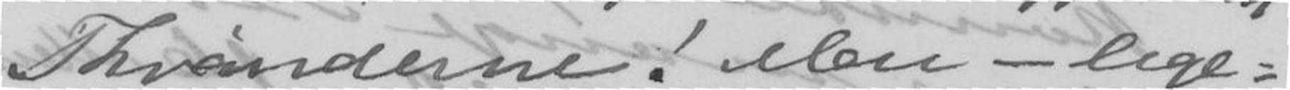Thrønderne! Men - lige-
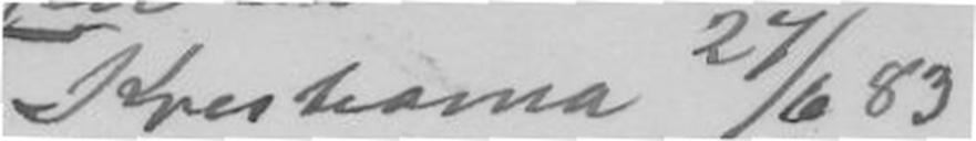Kristiania 27/6 83
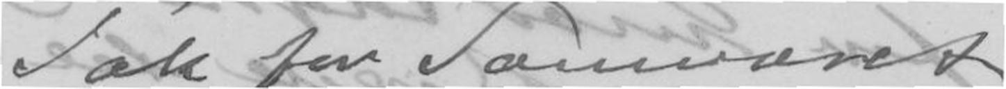Tak for Samværet
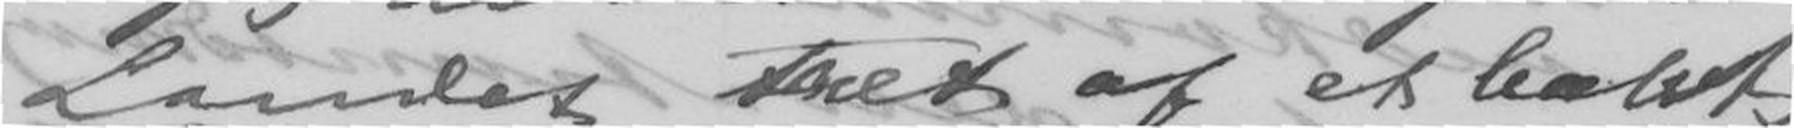Landet tret af et halvt
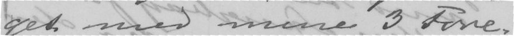get med mine 3 Fore-
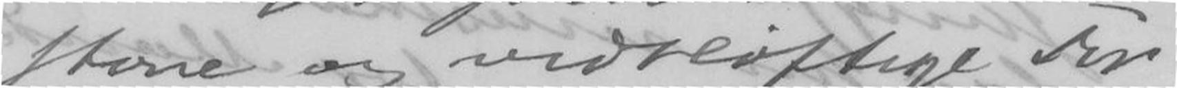store og vidtløftige For-
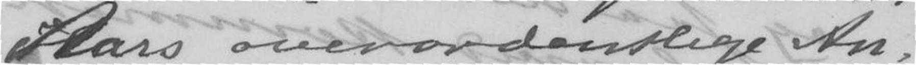Aars overordenrlige An-
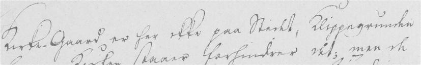Kirke-Gaard er her ikke paa Stedet, Klippegrunden
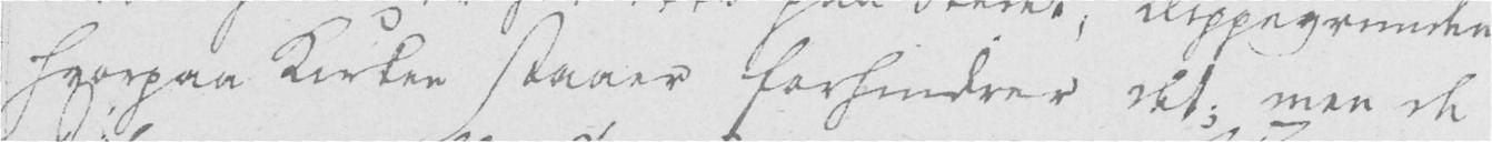hvorpaa Kirken staaer forhindrer det; men de
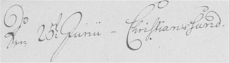Den 25te Junii Christianssund.
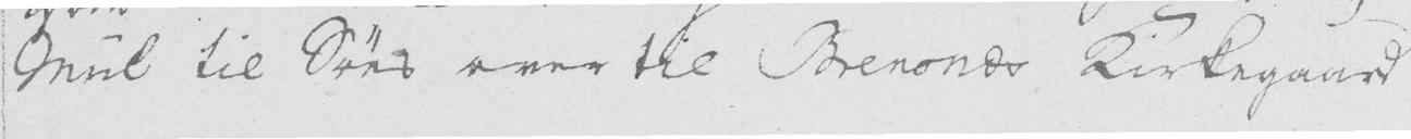Miil til Søes over til Brensnæs Kirkegaard
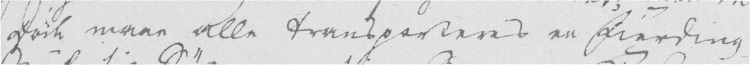Døde maae alle transporteres en Fierding
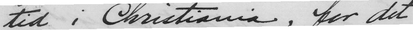tid i Christiania, for det
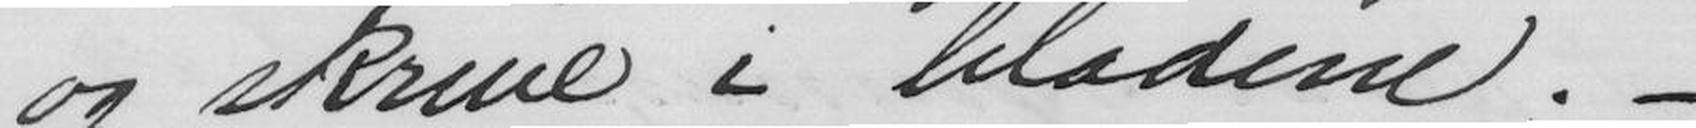og skrive i bladene. -
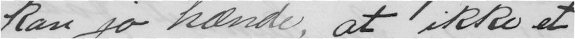kan jo hænde, at ikke et
